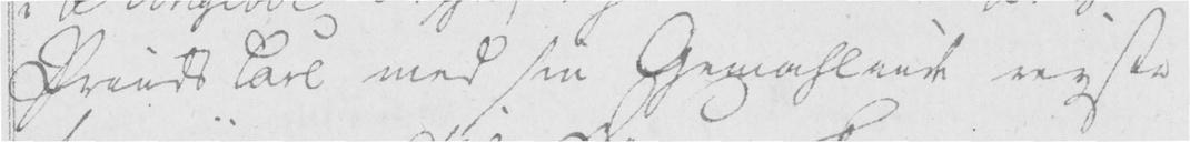Prinds Carl med sin Gemahlinde reyste
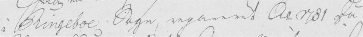i Ringeboe Sogn, repareret Aa 1781 Da
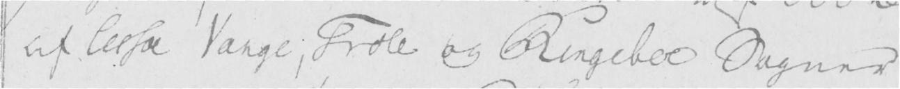af Lessa Vangl, Frole og Ringeboe Sogner
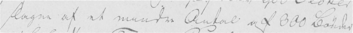slagne af et mindre Antal af 300 Bønder
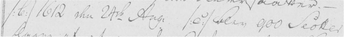/: b :/ 1612 den 24de Aug. /: c :/ blev 900 Scotter
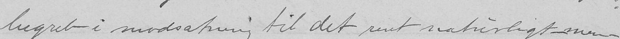begreb i modsætning til det rent naturligt men-
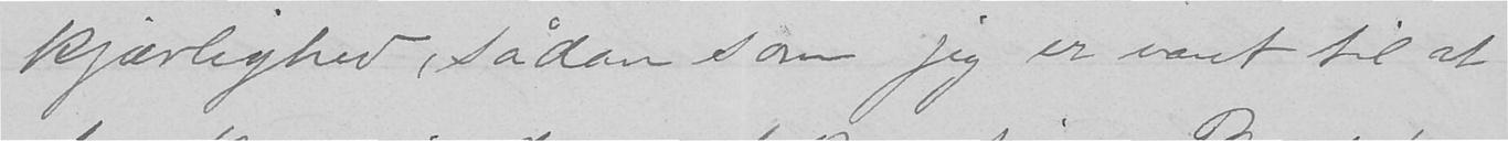kjærlighed, sådan som jeg er vant til at
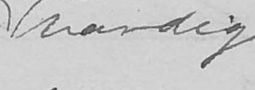værdig
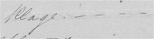klage. - - - -
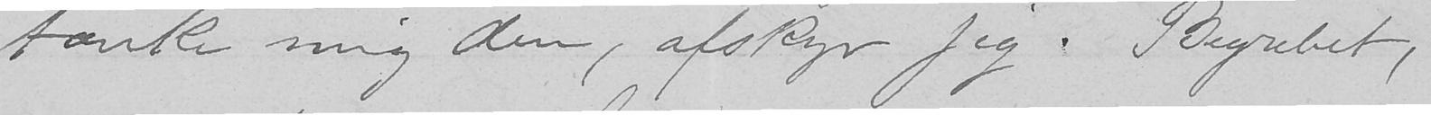tænke mig den, afskyr jeg. Begrebet,
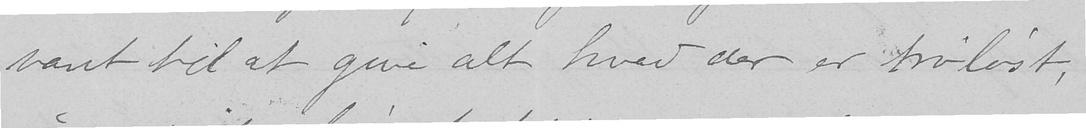vant til at give alt hvad der er troløst,
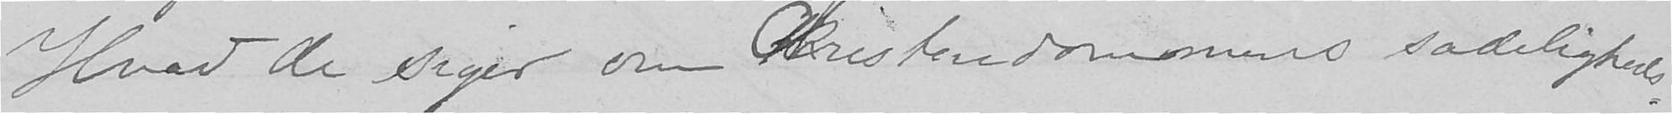Hvad de siger om Kristendommens sædeligheds-
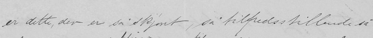er dette, der er så skjønt, så tilfredsstillende, så
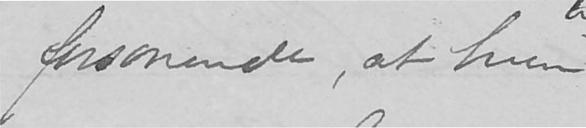forsonende, at hun
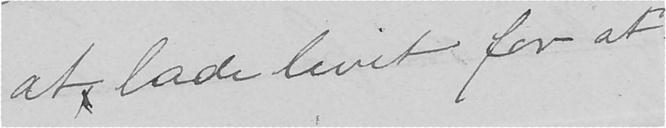at lade livet for at
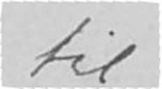til
